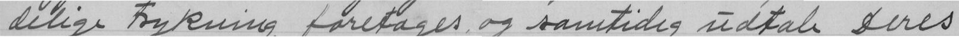delige Trykning foretages og samtidig udtale Deres
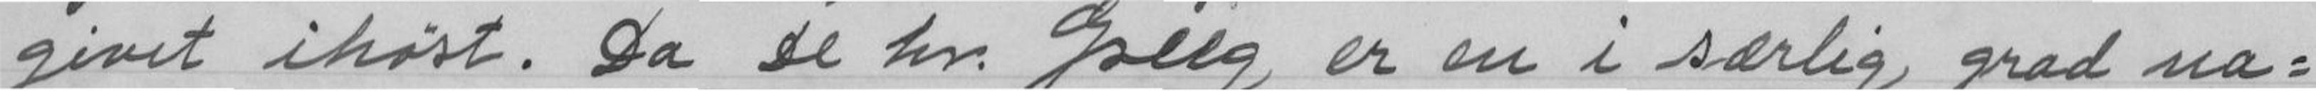givet ihøst. Da De hr. Grieg er en i særlig grad na-
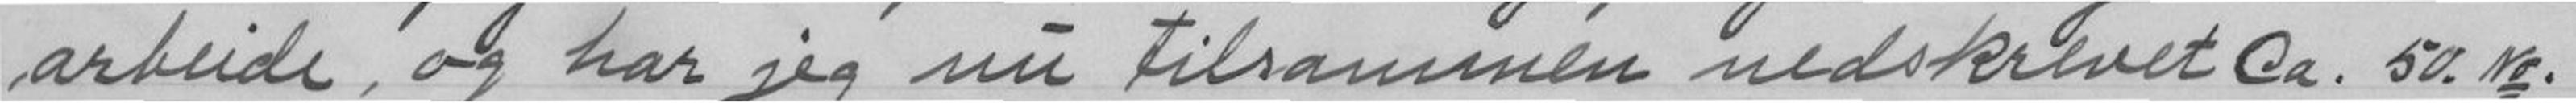arbeide, og har jeg nu tilsammen nedskrevet Ca. 50. No.
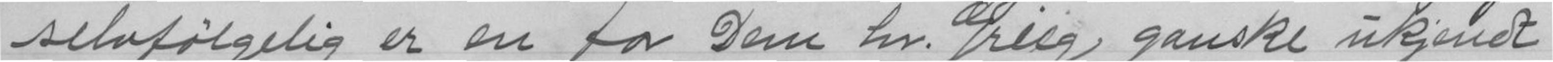selvfølgelig er en for Deres hr. Grieg ganske ukjendt
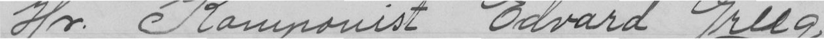Hr. Komponist Edvard Grieg
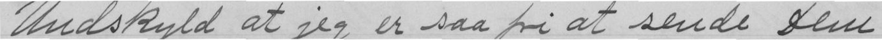Undskyld at jeg er saa fri at sende Dem
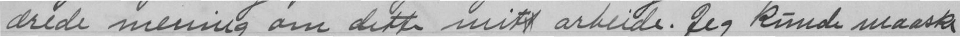ærede mening om dette mitt arbeide. Jeg kunde maaske
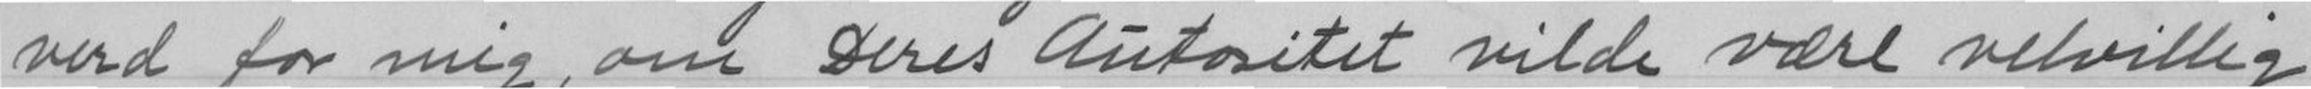verd for mig, om Deres Autoritet vilde være velvillig
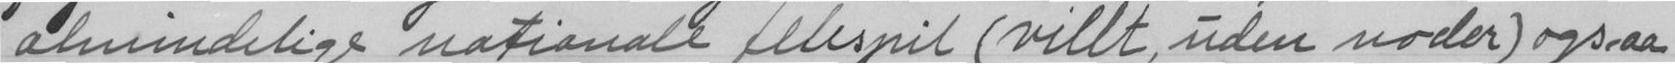almindelige nationale felespil (villt, uden noder) ogsaa
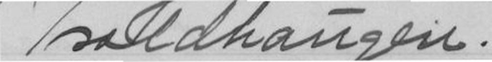Troldhaugen.
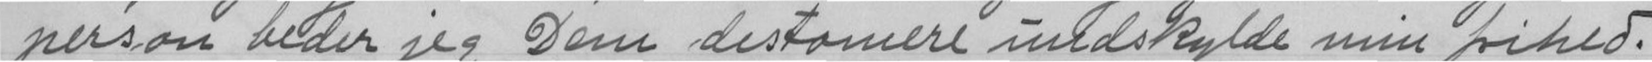person beder jeg Dem destomere undskylde min frihed.
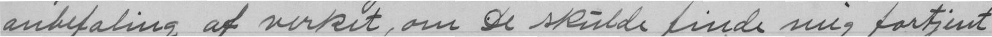anbefaling af verket, om De skulde finde mig fortjent
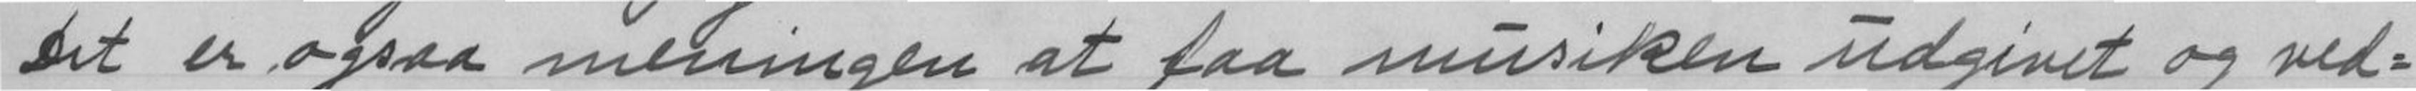Det er ogsaa meningen at faa musiken udgivet og ved-
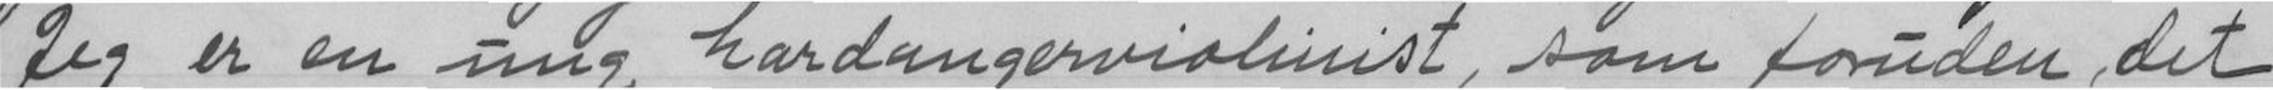Jeg er en ung hardangerviolinist, som foruden det
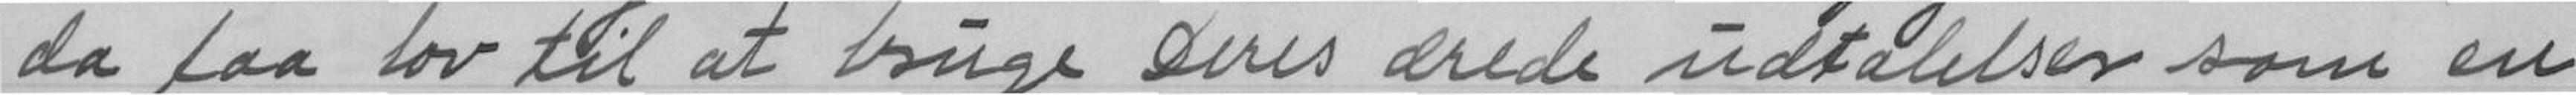da faa lov til at bruge Deres ærede udtalelser som en
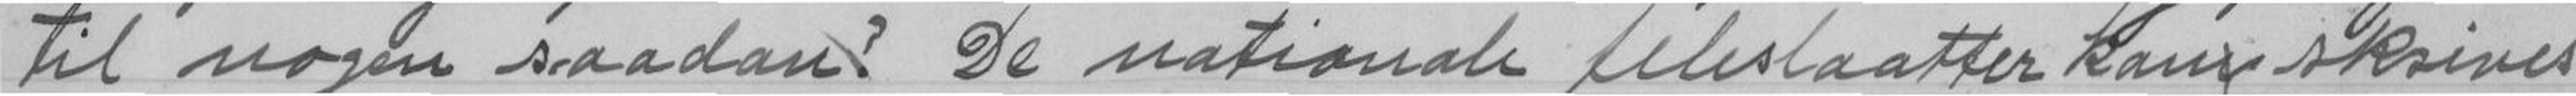til nogen saadan? De nationale feleslaatter kan skrives
